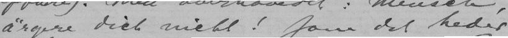ärgere dich nicht! som det heder
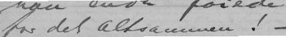for det Altsammen! -
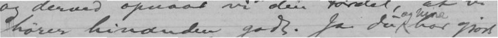hører hindanden godt. Ja Du har gjort
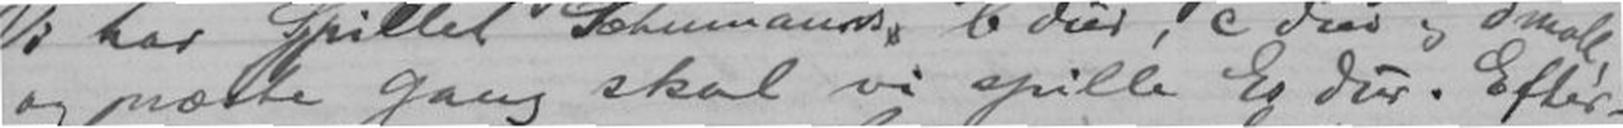og næste Gang skal vi spille Es dur. Efter-
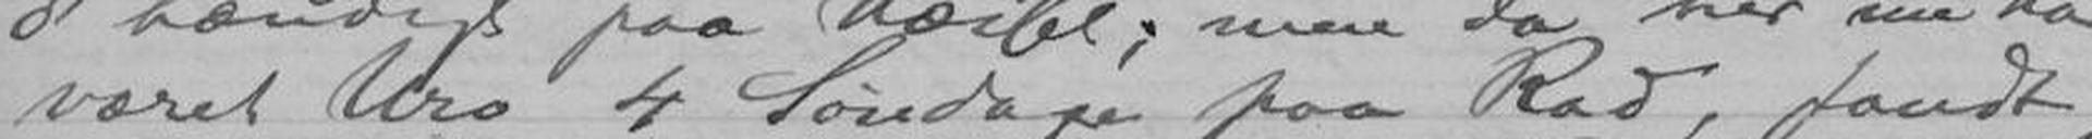været Uro 4 Søndage paa Rad, fandt
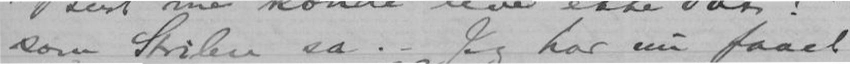som Strilen sa. - Jeg har nu faaet
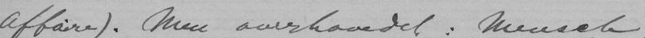Affaire). Men overhovedet: Mensch,
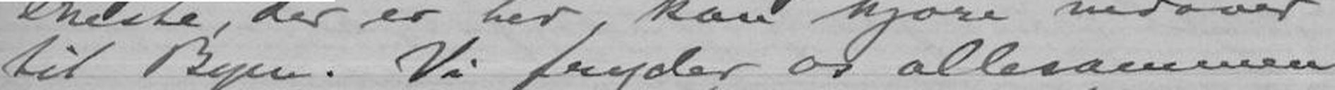til Byen. Vi fryder os allesammen
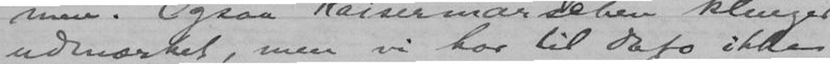udmærket, men vi har til Dato ikke
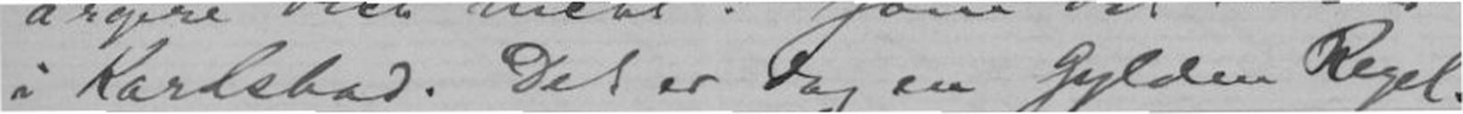i Karlsbad. Det er dog en Gylden Regel.
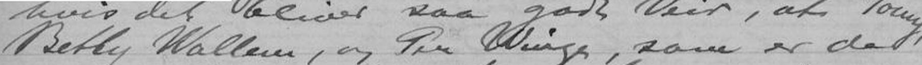Betty Wallem, og Per Winge, som er de
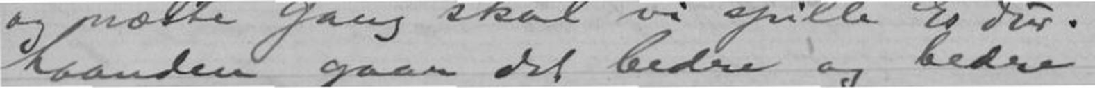haanden gaar det bedre og bedre;
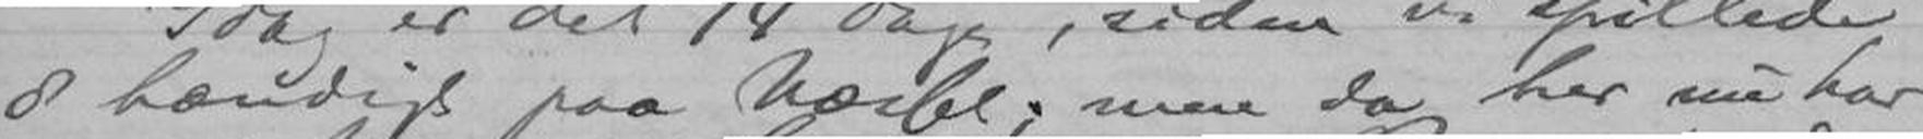8 hændigt paa Næsset; men da her nu har
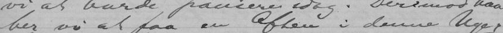ber vi at faa en Aften i denne Uge,
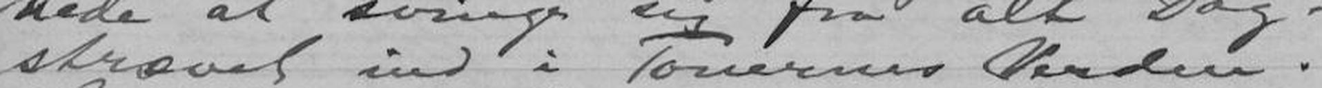strævet ind i Tonernes Verden.
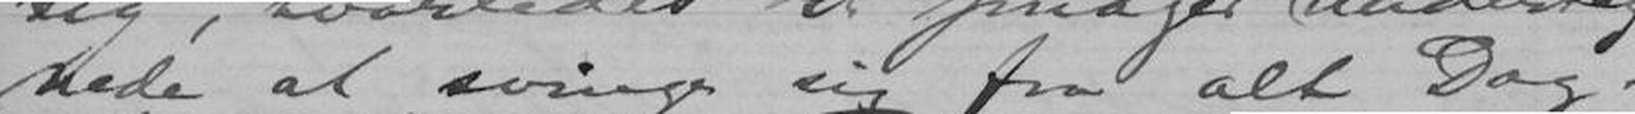nede at svinge sig fra alt Dag-
